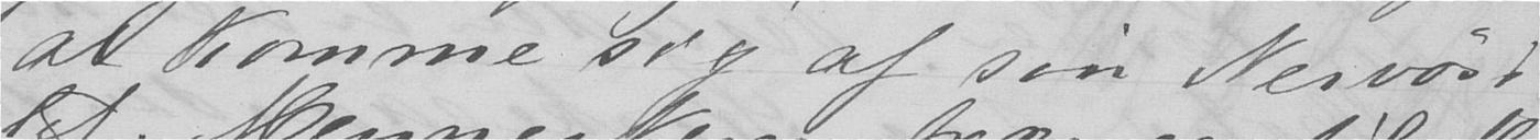at komme sig af sin Nervøsi-
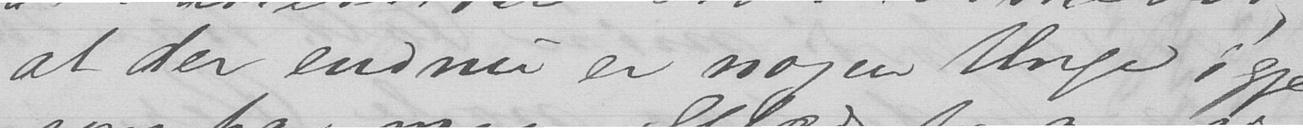at der endnu er nogen Unge igjen
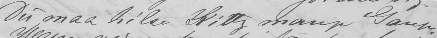Du maa hilse Kitty mange Gange.
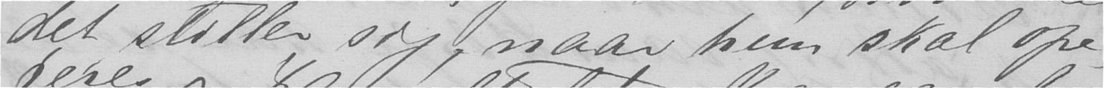det stiller sig, naar hun skal ope-
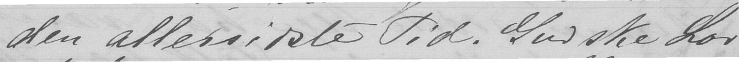den allersidste Tid. Gud ske Lov,
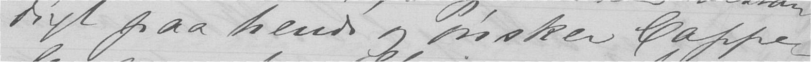digt paa hende og ønsker Cappe-
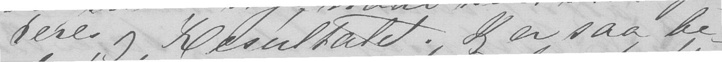reres, og Resultatet. Jeg er saa be-
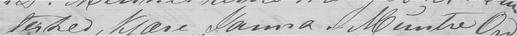terhed, kjære Janna. Muntre Ord
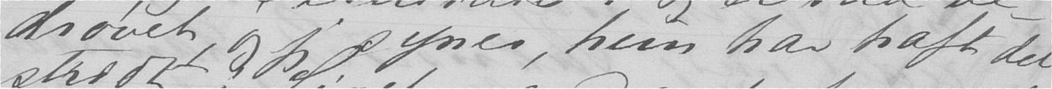drøvet, og jeg synes, hun har haft det
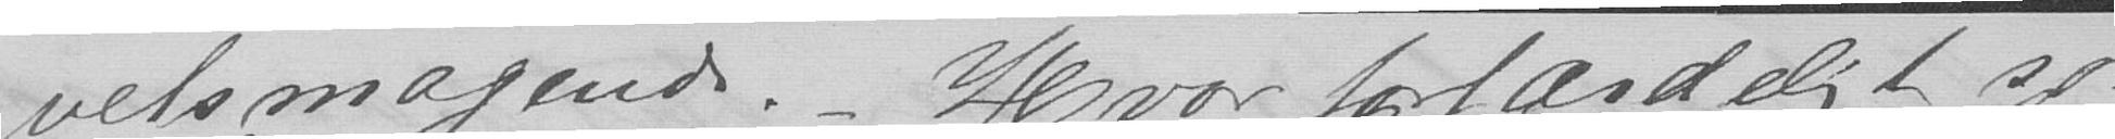velsmagende. - Hvor forfærdeligt sør-
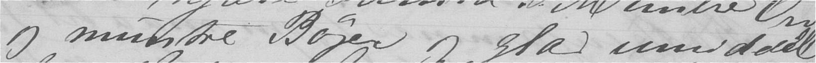og muntre Bøger og glad umiddel-
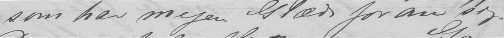som har megen Glæde foran sig.
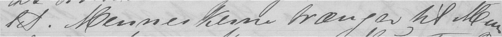tet. Menneskerne trænger til Mun-
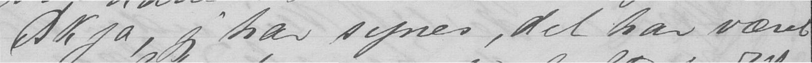Ak ja, jeg har synes, det har været
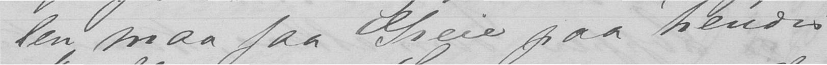len maa faa Greie paa hendes
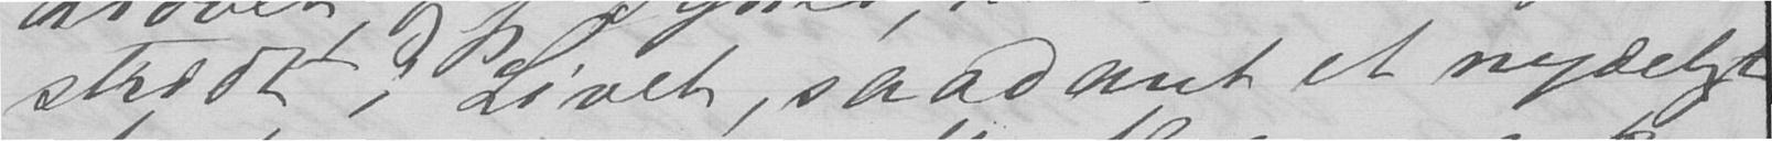stridt i Livet, saadant et nydeligt
